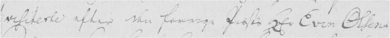visiterte efter den forrige Præsts Hr Even Olsens
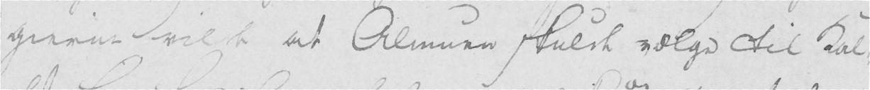gierne vilde at Almuen skulde vælge til Kal-
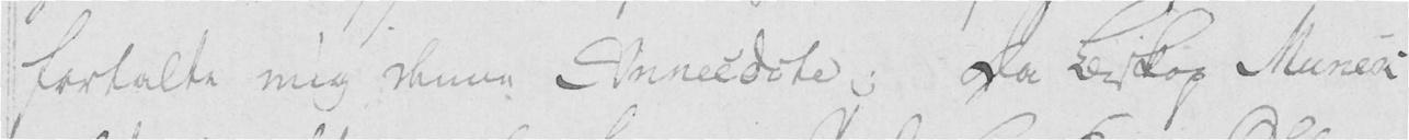fortalte mig denne Annecdote; Da Biskop Munck
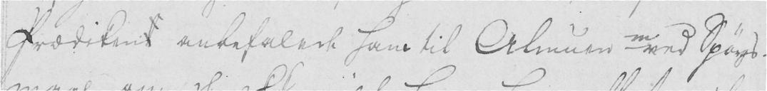Prædiken anbefalede ham til Almuen med Spørgs-
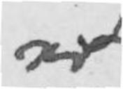er
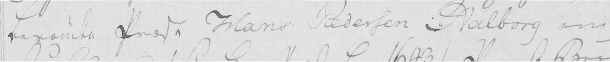berømte Præst Hans Pedersen Valborg en
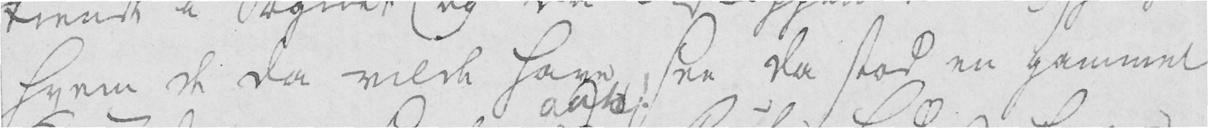hvem de da vilde have, see da stod en gammel
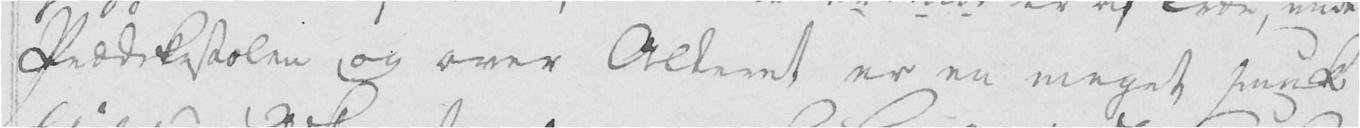Prædikestolen og over Alteret er en meget smuck
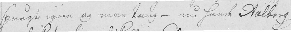spurgte igien og man taug - nu havde Aalborg
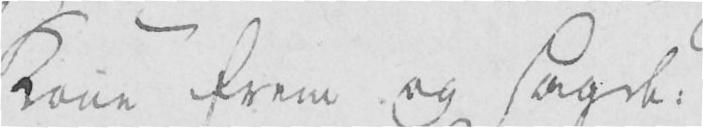Kone frem og sagde:
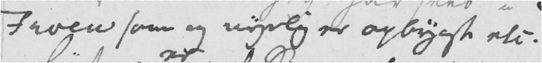Inven som og nyelig er opbygt alt.
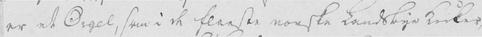er et Orgel, som i de fleeste norske Landsbye Kirker
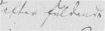efter fuldendt
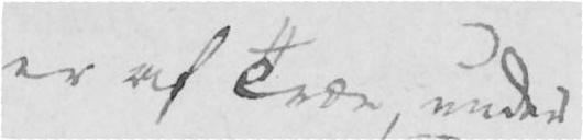er af Træe, under
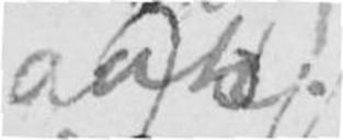aah!
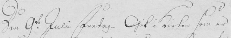Den 9de Julii Fredag - Gik i Kirken som er
